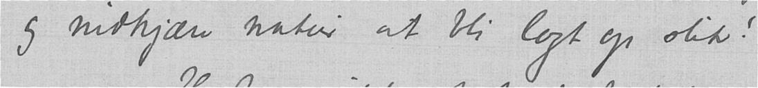og nidkjære natur at bli lagt op slik!
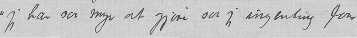"jeg har saa mye at gjøre saa jeg ingenting faar
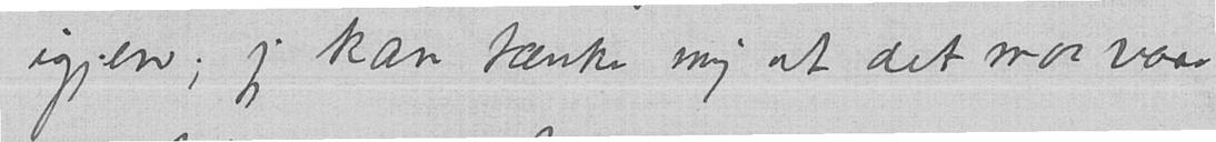igjen; jeg kan tænke mig at det maa være
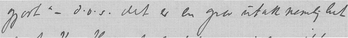gjort" - d.v.s. det er en grov utaknemlighet
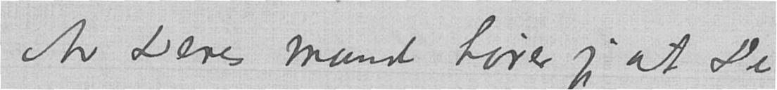Av Deres mand hører jeg at De
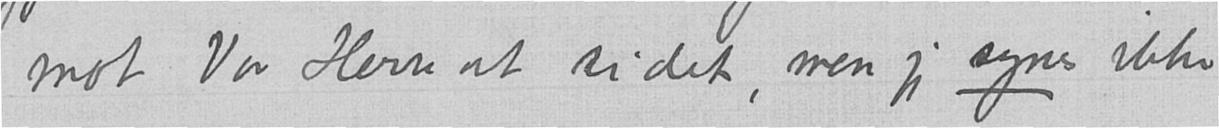mot Vor Herre at si det, men jeg synes ikke
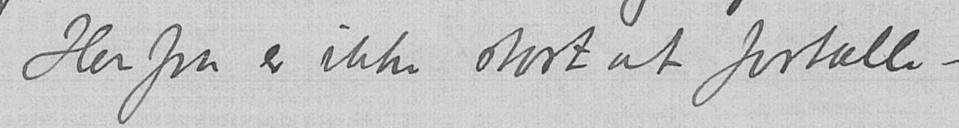Herfra er ikke stort at fortælle –
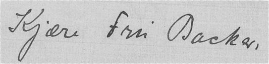Kjære Fru Backer,
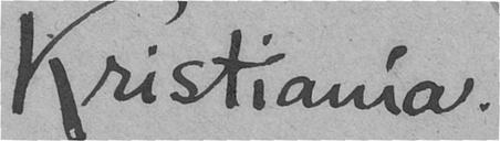Kristiania.
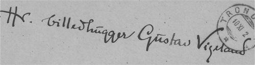Hr. billedhugger Gustav Vigeland
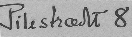Pilestrædet 8
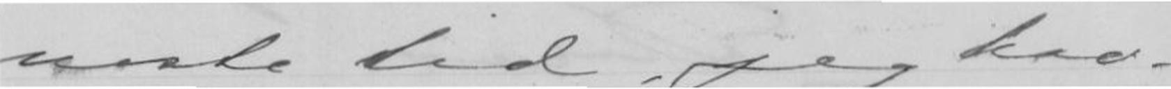neste tid jeg hav-
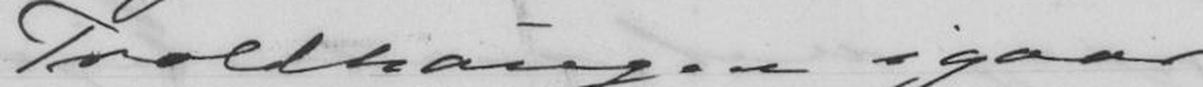Troldhaugen igaar
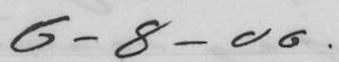6 - 8 - 06.
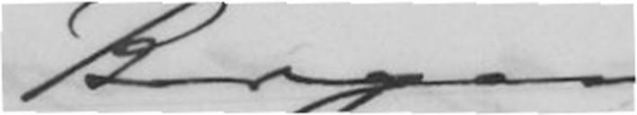Bergen
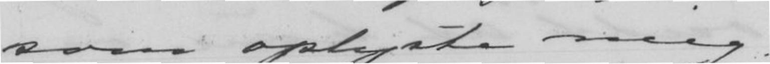som oplyste mig
